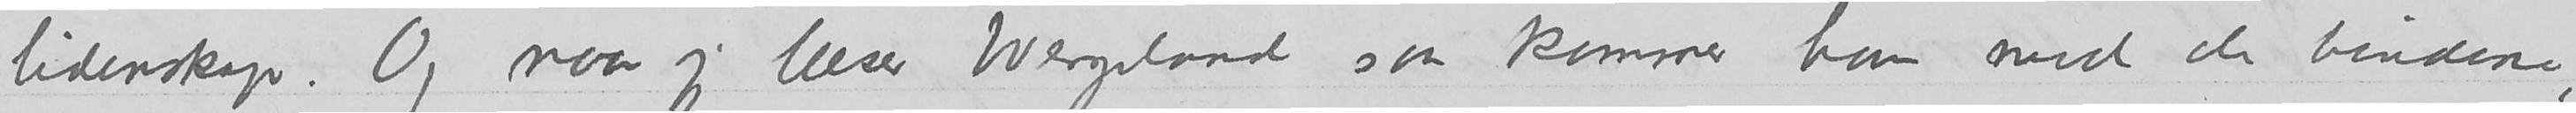lidenskap. Og naar jeg læser Wergeland saa kommer han med de bindene,
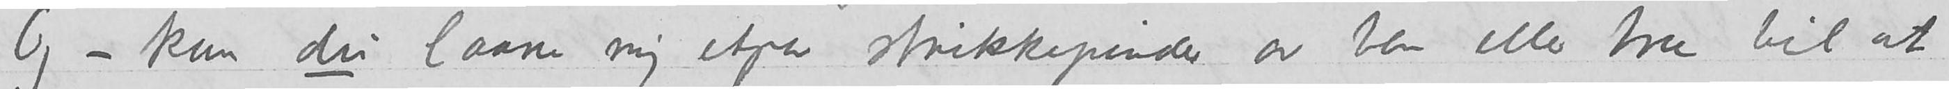Og – kan du laane mig etpar strikkepinner av ben eller tre til at
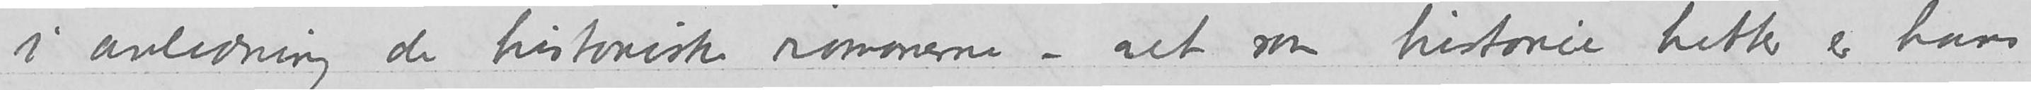med i anledning de historiske romanerne – alt som historie hetter er hans
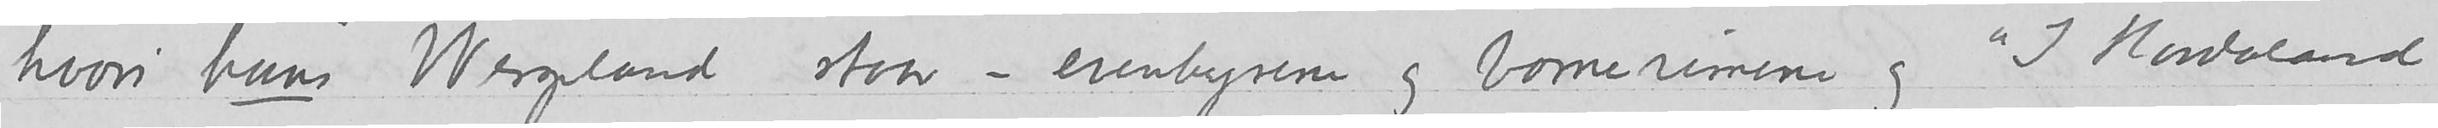hvori hans Wergeland staar – eventyrene og børnerimene og «I Hordaland
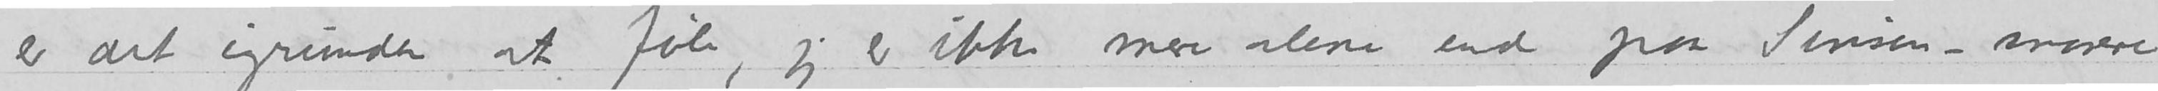er det igrunden at føle, jeg er ikke mere alene end paa Sinsen – snarere
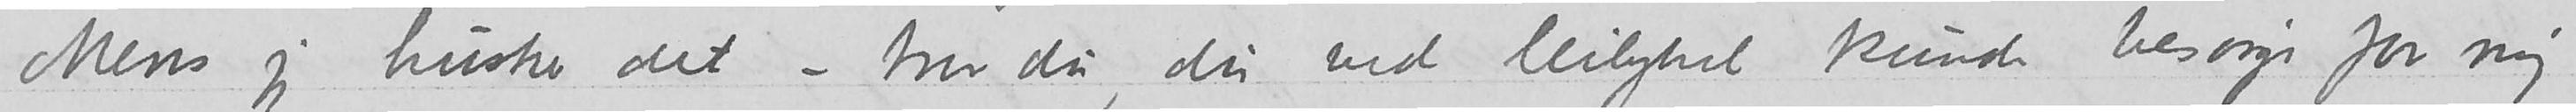Mens jeg husker det – tror du, du ved leilighet kunde besørge for mig
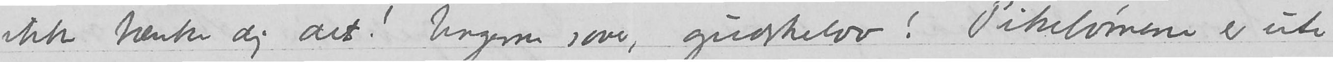ikke tænke dig det! Ungerne sover, gudskelov! Pikebørnene er ute
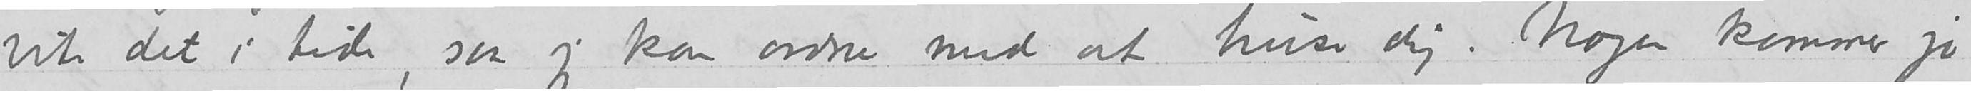vite det i tide, saa jeg kan ordne med at huse dig. Nogen kommer jo
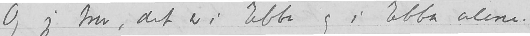Og jeg tror det er i Ebba og i Ebba alene.
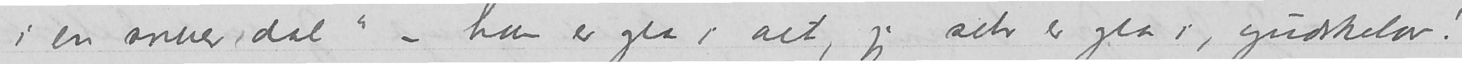i en annen dal» - han er gla i alt, jeg selv er gla i, gudskelov!
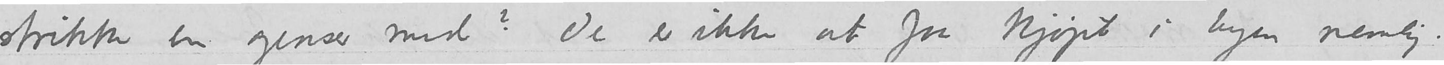strikke en genser med? De er ikke at faa kjøpt i byen nemlig.
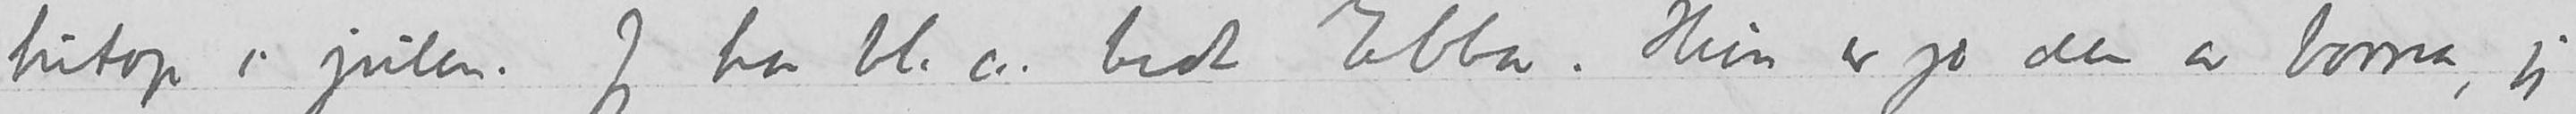hitop i julen. Jeg har bl.a. bedt Ebba. Hun er jo den av barna, jeg
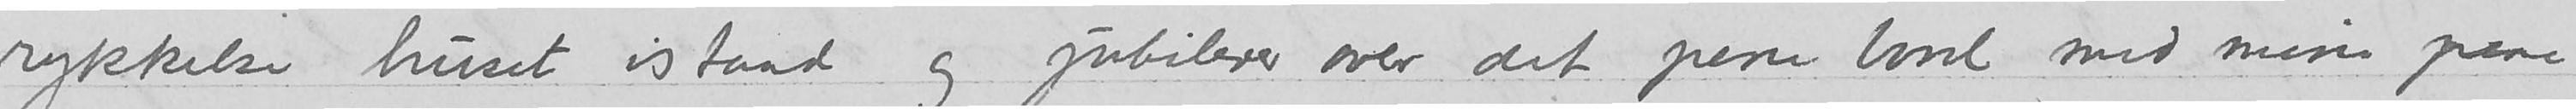rykkelse huset istand og jubilerer over det pene bord med mine pene
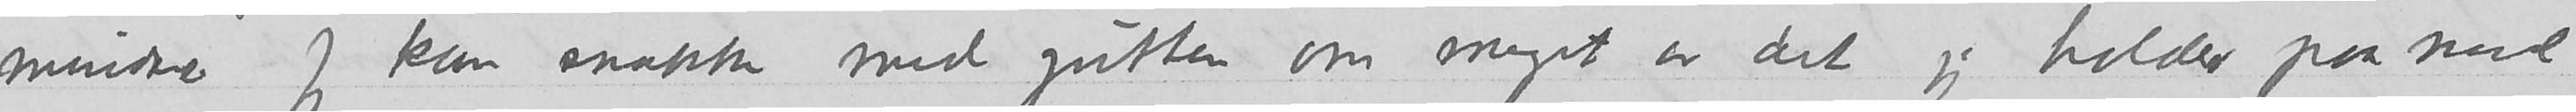mindre Jeg kan snakke med gutten om meget av det jeg holder paa
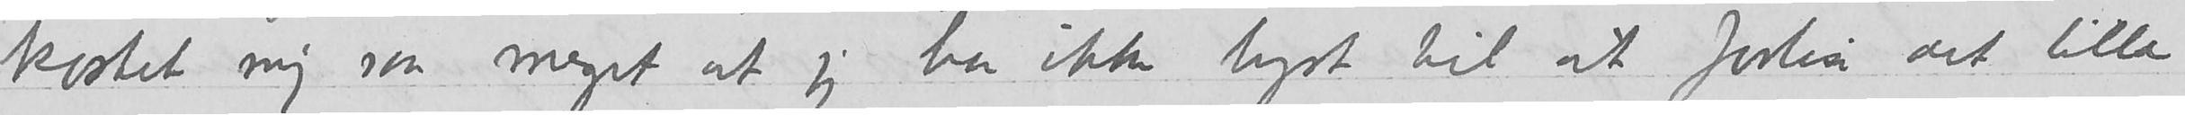kostet mig saa meget at jeg har ikke lyst til at forlise det lille
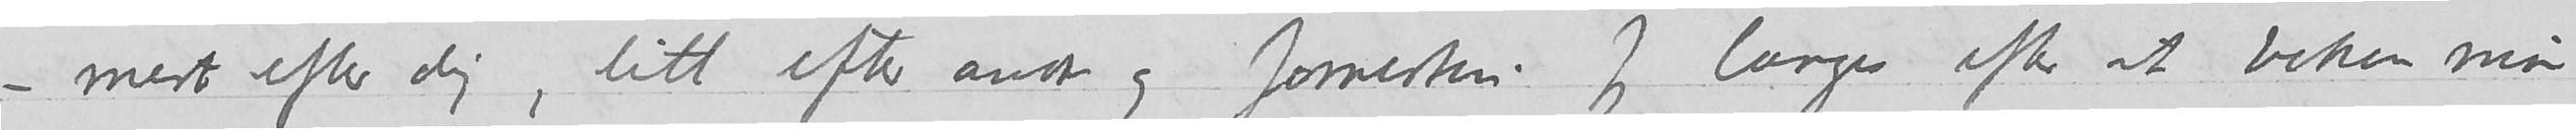mest efter dig, litt efter andre og forresten. Jeg længes efter at boken min
